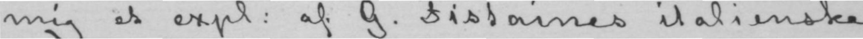mig et expl: af G. Fistaines italienske
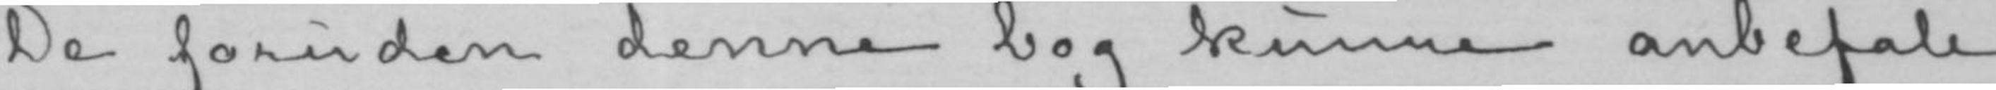De foruden denne bog kunne anbefale
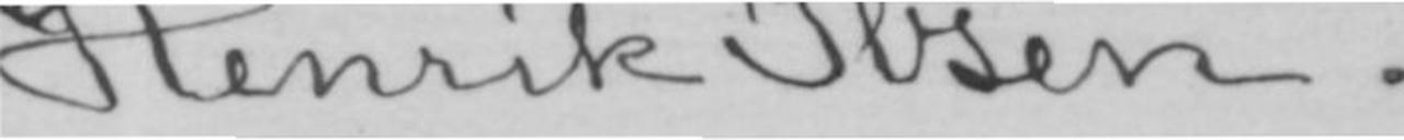Henrik Ibsen.
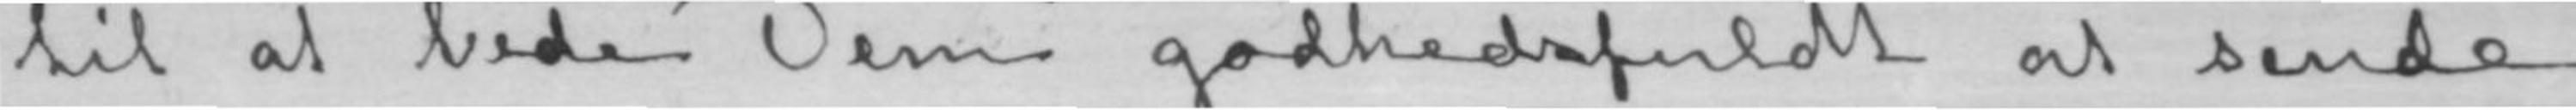til at bede Dem godhedsfuldt at sende
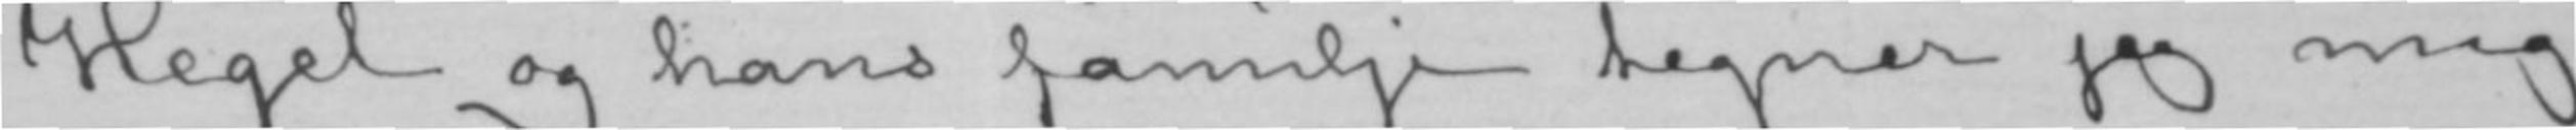Hegel og hans familje tegner jeg mig
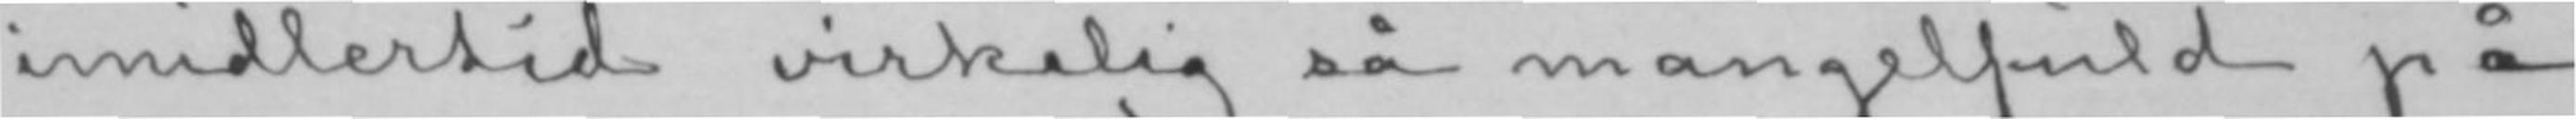imidlertid virkelig så mangelfuld på
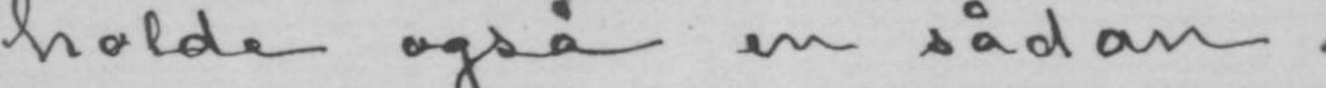holde også en sådan.
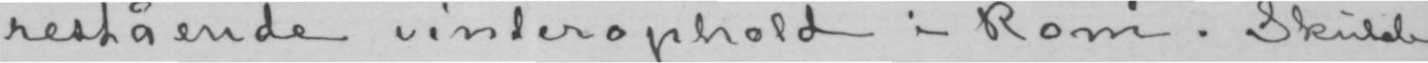restående vinterophold i Rom. Skulde
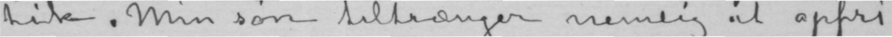tik. Min søn tiltrænger nemlig at opfri-
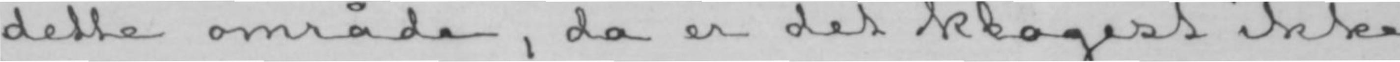dette område, da er det klogest ikke
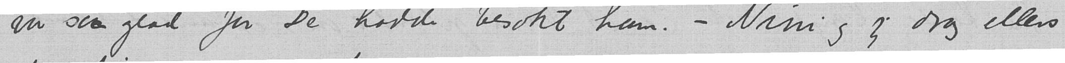var saa glad for De hadde besøkt ham. – Nini og jeg drog ellers
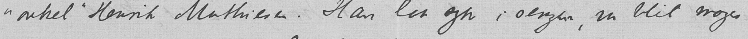«onkel» Henrik Mathiesen. Han laa syk i sengen, var blit mager
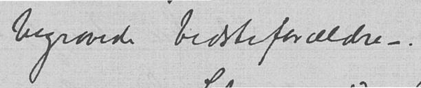begravede bedsteforældre –.
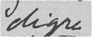digre
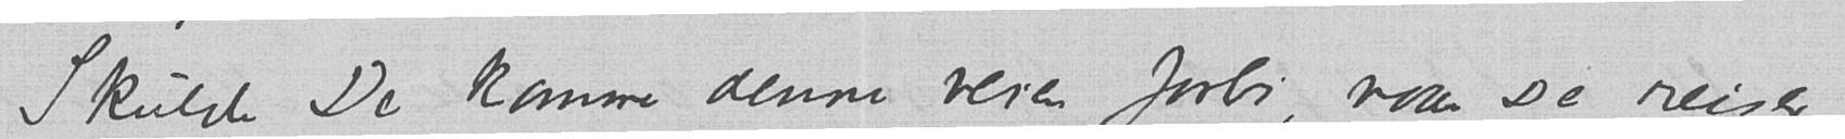Skulde De komme denne veien forbi, naar De reiser
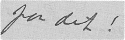paa det!
